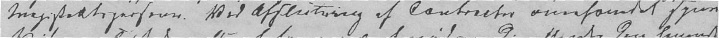Magistratspersoner. Ved Afslutning af Contracter overhovedet synes
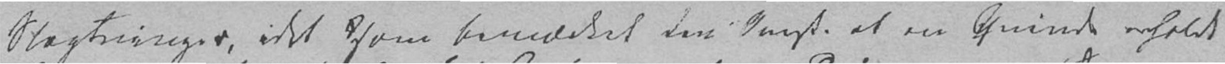Slægtninger, idet som bemærket den Omst. at en Qvinde erholdt
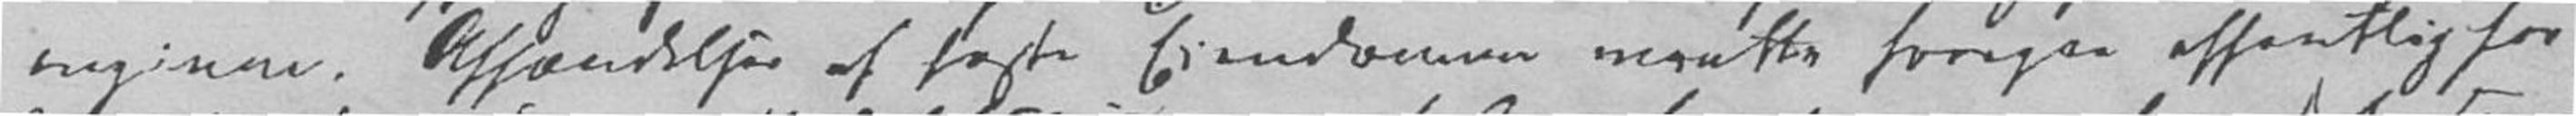angivne. Afhændelser af faste Eiendomme maatte foregaa offentlig for
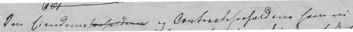Om Eiendoms og Contractsforholdene have vi
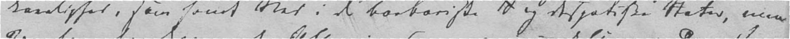kaarlighed, som fandt Sted i de barbariske og despotiske Stater, maa
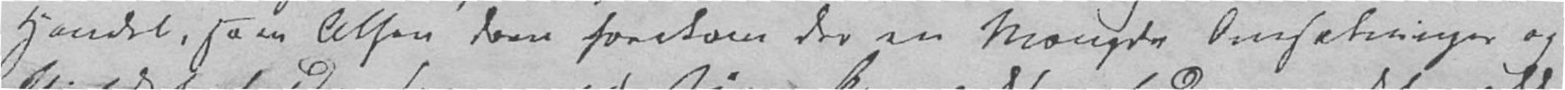Handel, som Athen drev forekom der en Mængde Omsætninger og
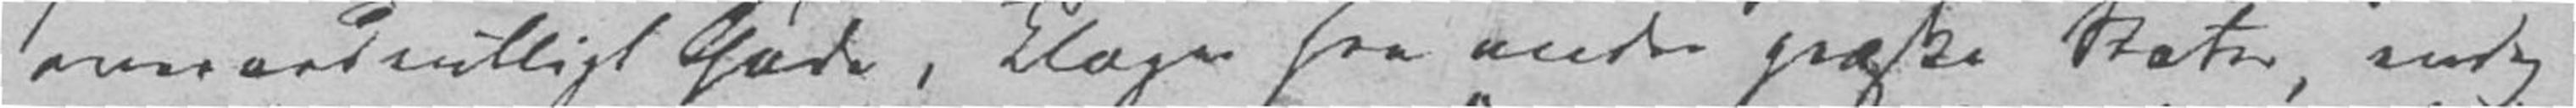overordentligt Gode, Klager fra andre græske Stater, endog
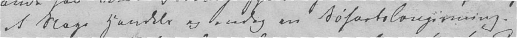et Slags Handels og endog en Søfartslovgivning.
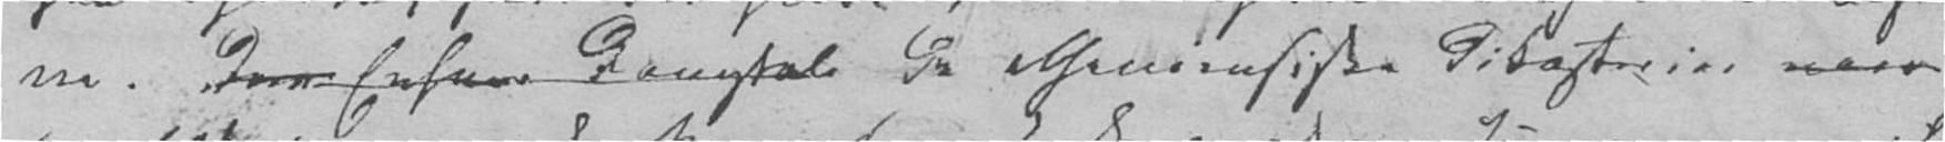ne. De athenienske Dikatorier
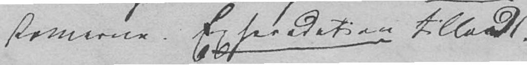Romerne. Experedation tilladt
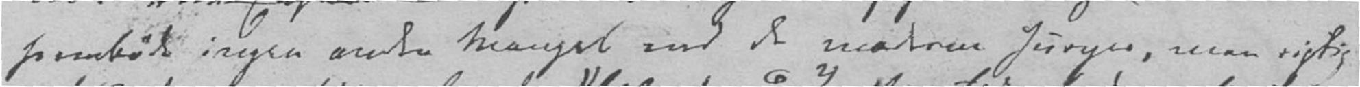frembøde ingen anden Mangel end de moderne Juryes, men rigtig
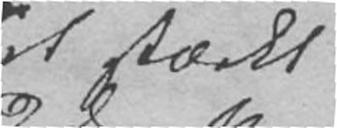et stærkt
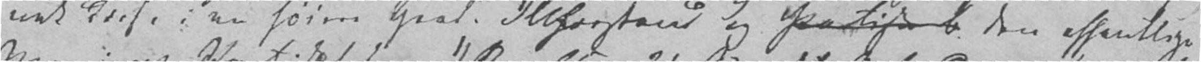nok disse i en høiere Grad. Illforstand og den offentlige
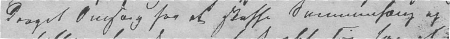draget Omsorg for at skaffe Sammenhæng og Orden ind i dem,
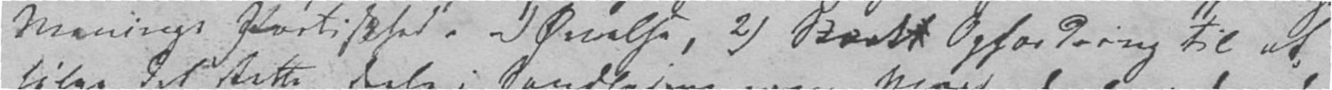Menings Partiskhed, 1) Øvelse, 2) Opfordring til at
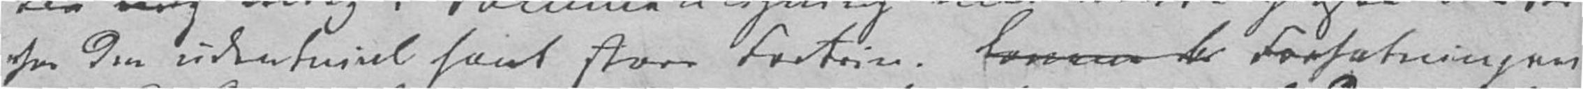har den udentvivl havt store Fortrin. Forfatningen
